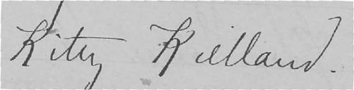Kitty Kielland.
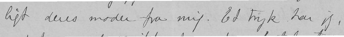ligt deres moder fra mig. Et tryk har jeg,
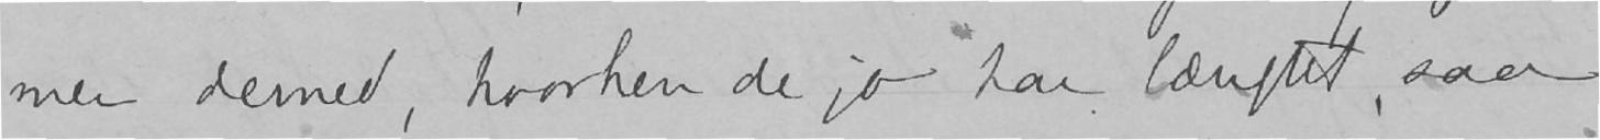mer derned, hvorhen de jo har længtet, saa
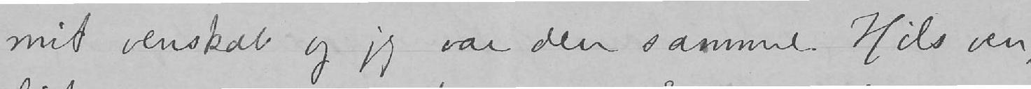mit venskab og jeg var den samme. Hils ven-
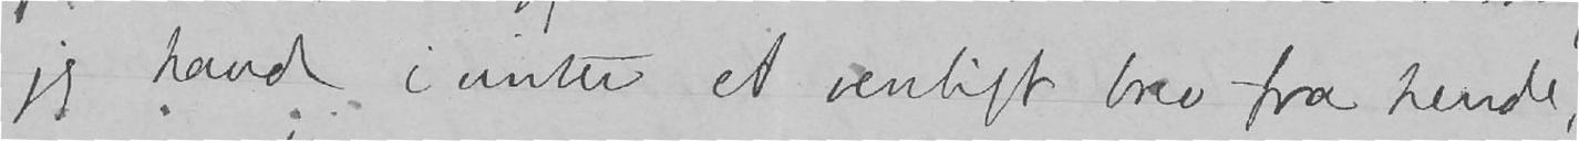jeg havde i vinter et venligt brev fra hende,
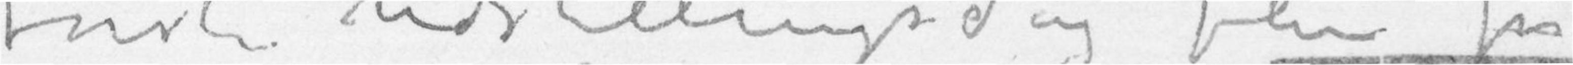første Udstillingsdag flere for
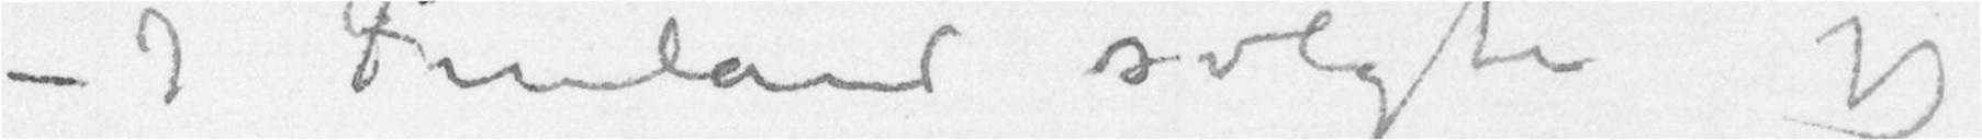– I Finland solgte jeg
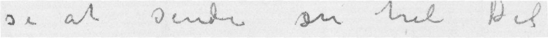se at sende en hel Del
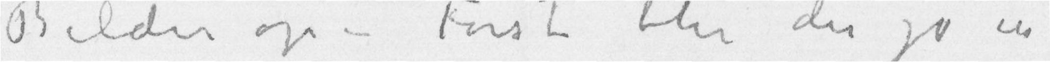Bilder op – Først blir der jo en
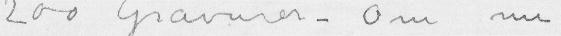200 Gravurer – Om nu
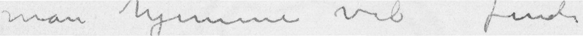man hjemme vil finde
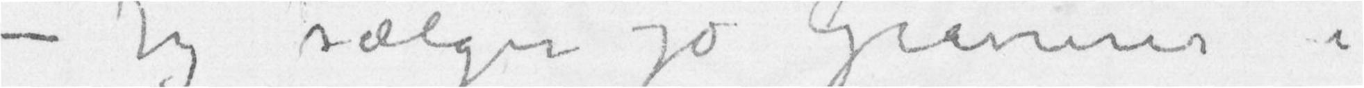– Jeg sælger jo Gravurer i
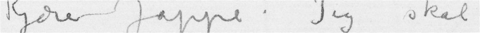Kjære Jappe! Jeg skal
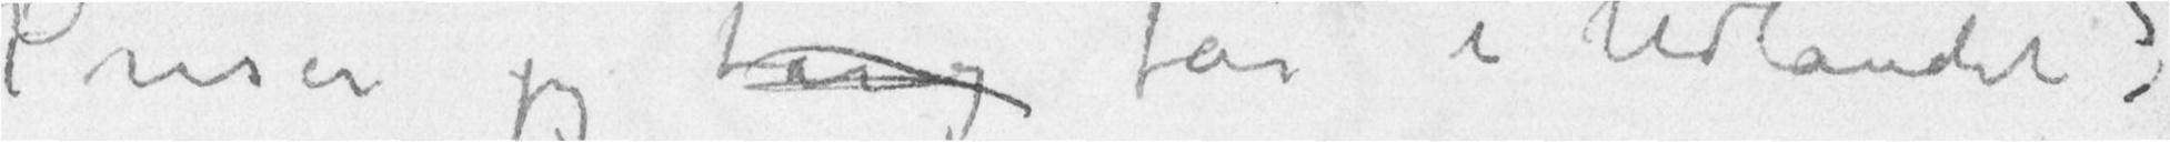Priser jegfår i Udlandet?
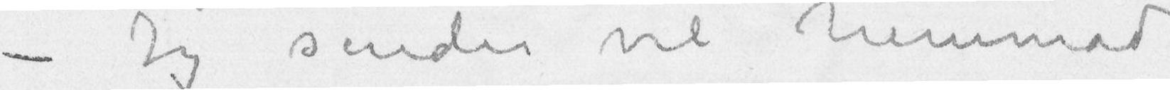– Jeg sender vel henimod
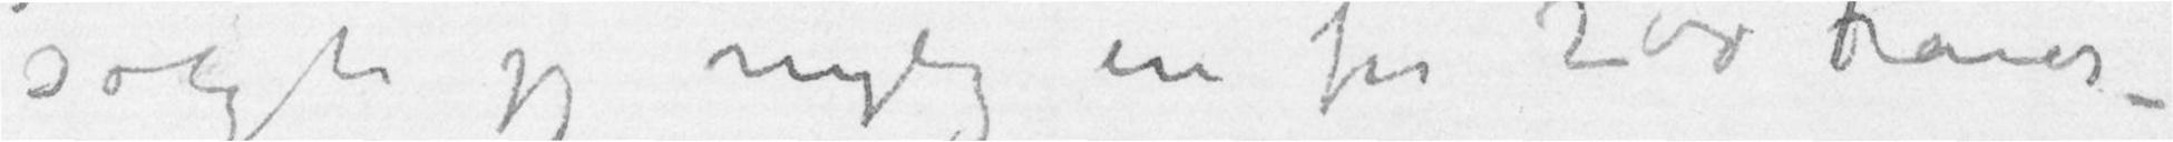solgte jeg nylig en for 200 Francs –
